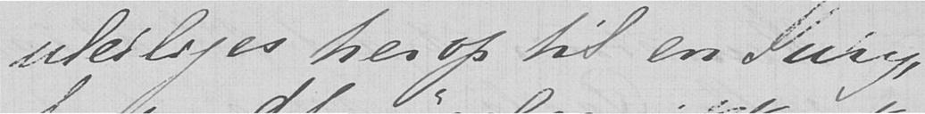uleiliges herop til en Jury,
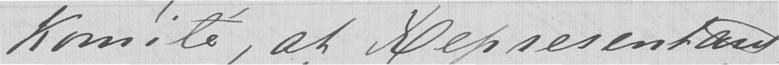komité, at Representant-
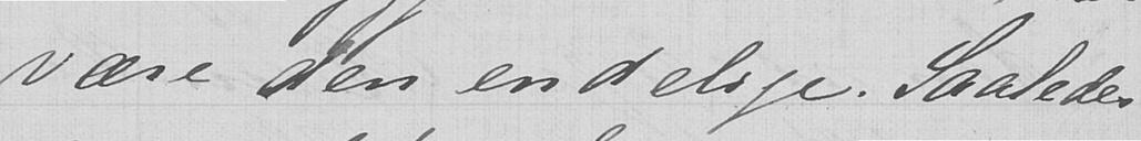være den endelige. Saaledes
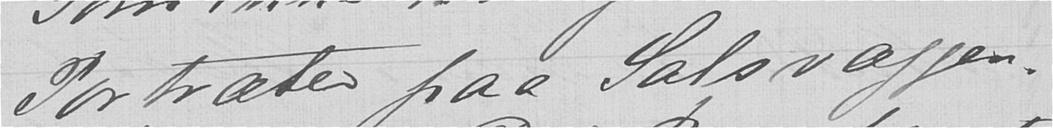Portræter paa Salsvæggen.
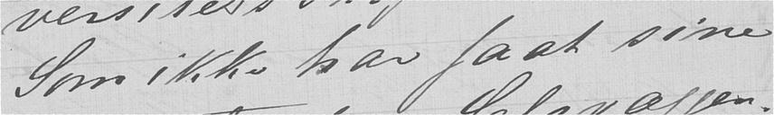Som ikke har faat sine
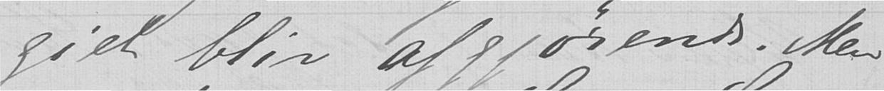giet blir afgjørende. Men
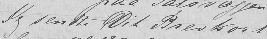Jeg sendte Dit Brevkort
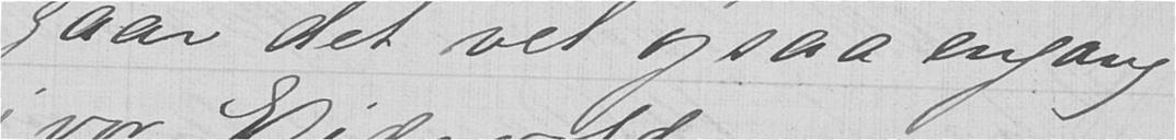gaar det vel ogsaa engang
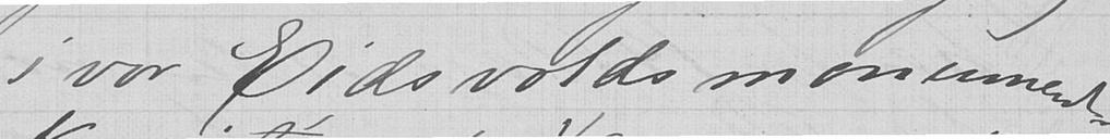i vor Eidsvolds monument-
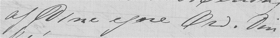af Dine egne Ord. Din
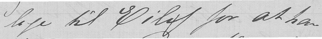lige til Eilif for at han
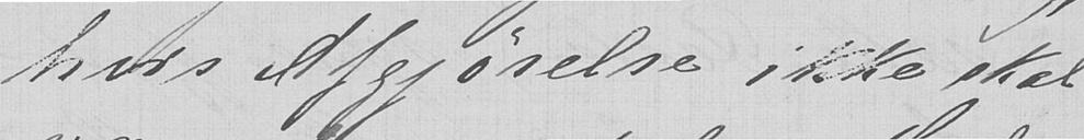hvis Afgjørelse ikke skal
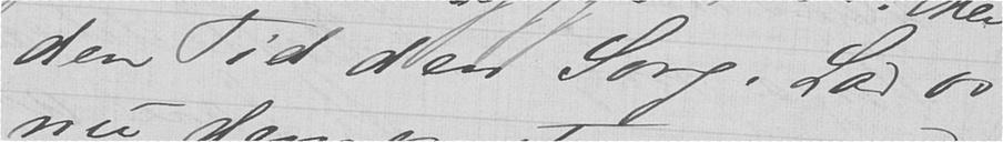den Tid den Sorg. Lad os
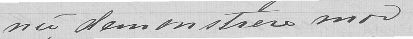nu demonstrere mod
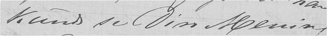kunde se Din Mening
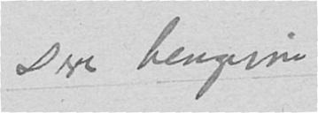Deres hengivne
